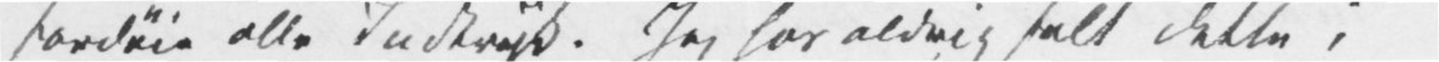fordøie alle Indtryk. Jeg har aldrig følt dette i
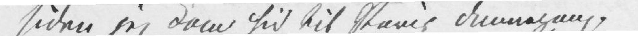siden jeg kom hid til Paris dennegang.
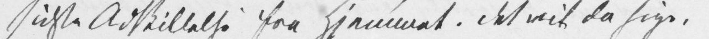sidste Adskillelse fra Hjemmet. Det vil da sige,
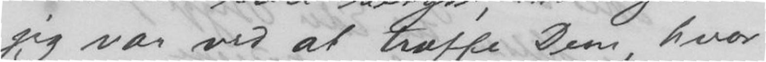jeg var ved at træffe Dem, hvor
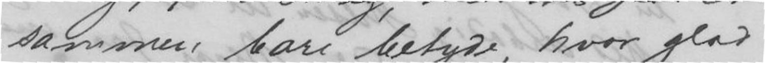sammen, bare betyde, hvor glad
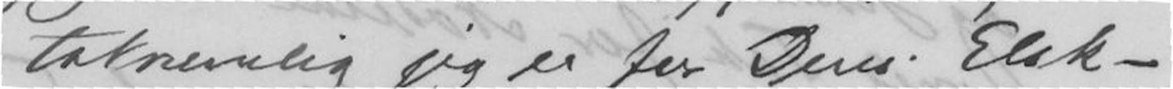taknemlig jeg er for Deres Elsk-
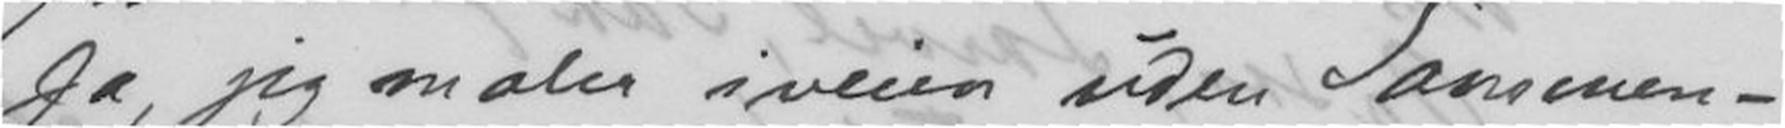Ja, jeg maler iveien uden Sammen-
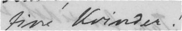fine Kvinder!
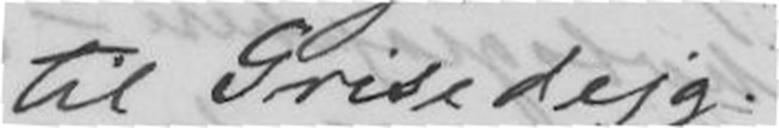til Grisedejg.
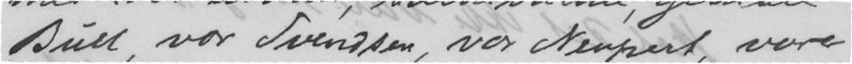Bull, vor Svendsen, vor Neupert, vore
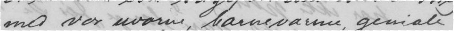med vor uvorne, barnevarme, geniale
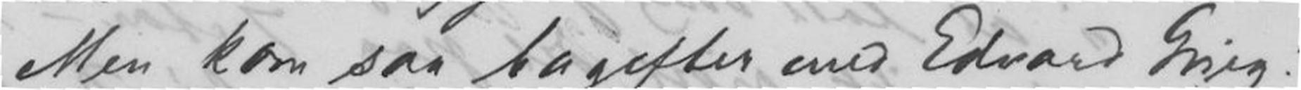Men kom saa bagefter med Edvard Grieg!
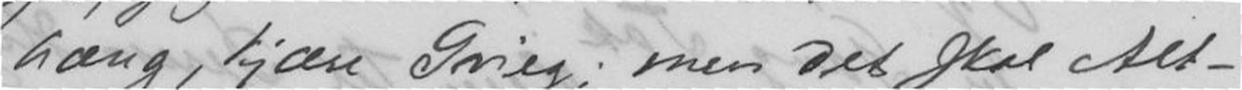hæng, kjære Grieg; men det skal Alt-
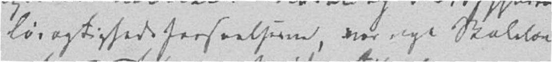Løsagtighedsforseelserne, vor nye Skolelov
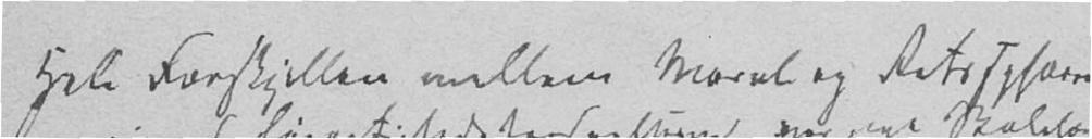Hele Forskjellen mellem Moral og Retssphære
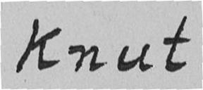Knut
